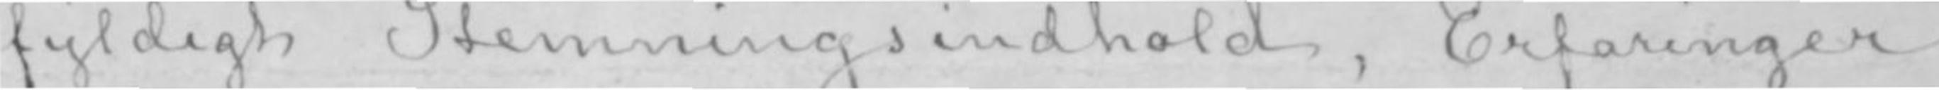fyldigt Stemningsindhold, Erfaringer
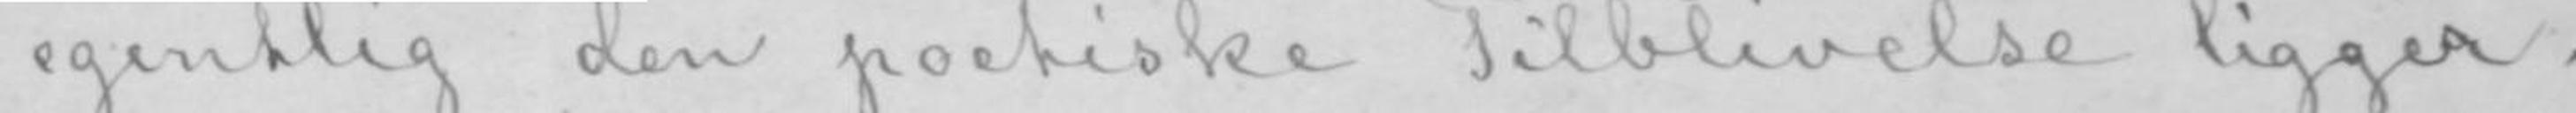egentlig den poetiske Tilblivelse ligger.
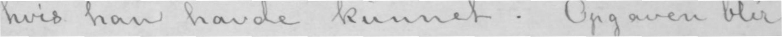hvis han havde kunnet. Opgaven blir
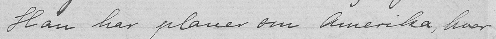Han har planer om Amerika, hvor
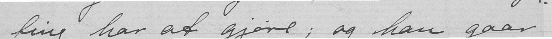ting har at gjøre; og han gaar
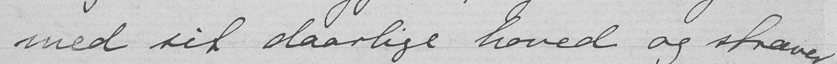med sit daarlige hoved og stræver
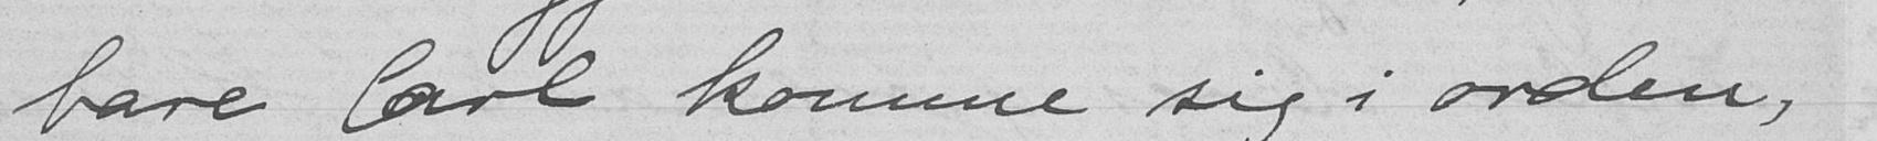bare Carl komme sig i orden,
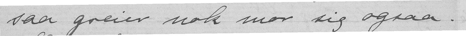saa greier nok mor sig ogsaa.
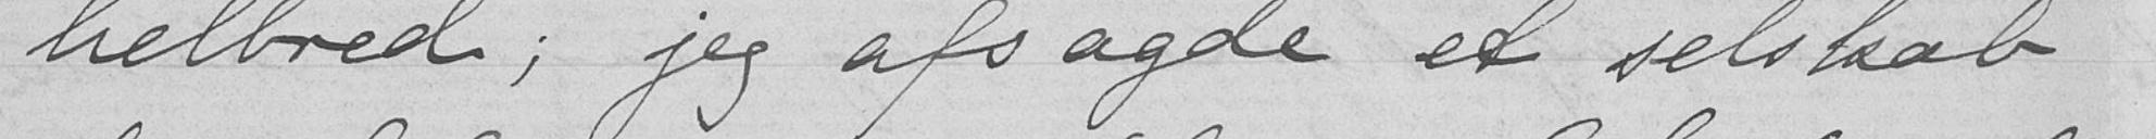helbred; jeg afsagde et selskab
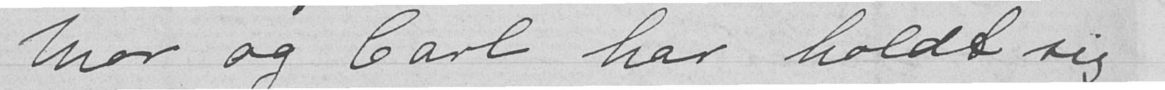Mor og Carl har holdt sig
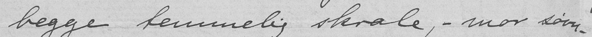begge temmelig skrale, - mor søvn-
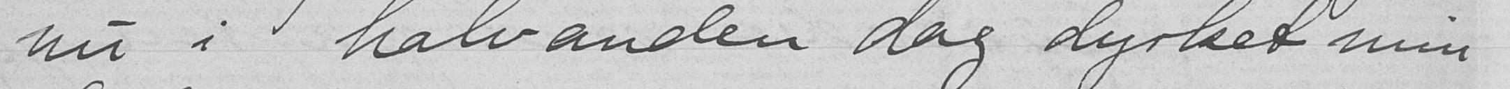nu i halvanden dag dyrket min
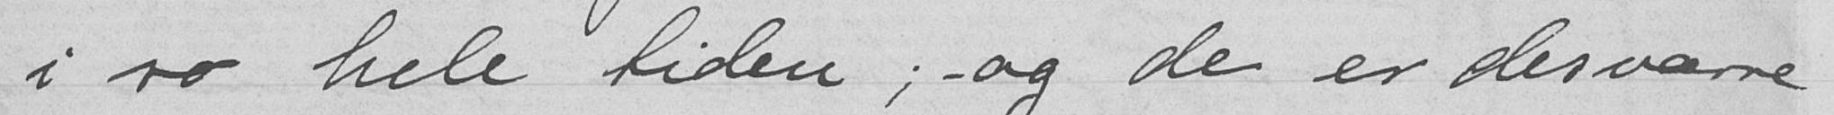i ro hele tiden; - og de er desværre
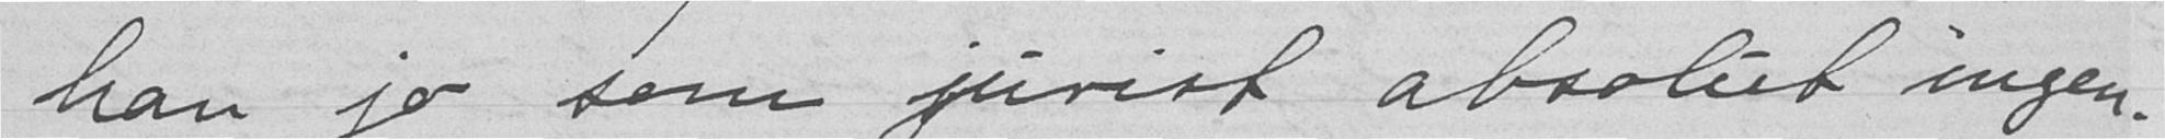han jo som jurist absolut ingen-
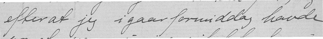efter at jeg igaar formiddag havde
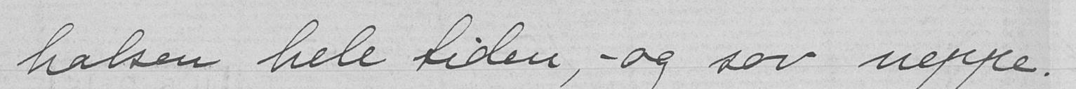halsen hele tiden, - og sov neppe.
